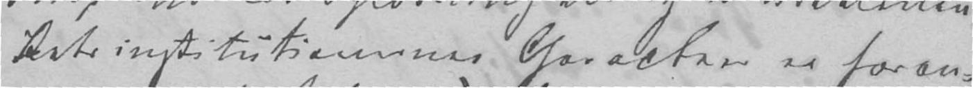Retsinstitutionernes Character er foran-
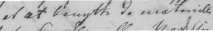af at benytte de materielle
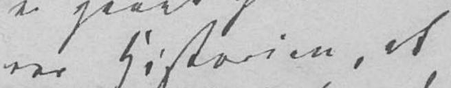rer Historien, at
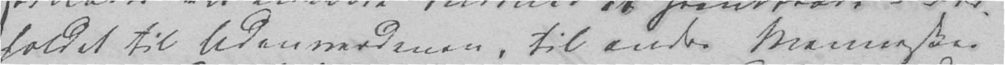holdet til Udenverdenen, til andre Mennesker
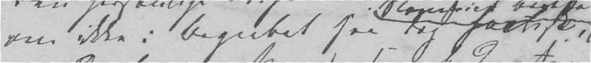om ikke i Begrebet saa dog factisk,
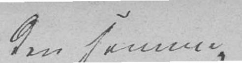den samme,
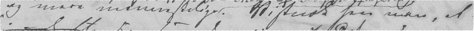og mere menneskelige. Vistnok seer man, at
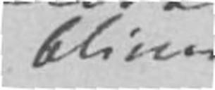bliver
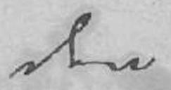den
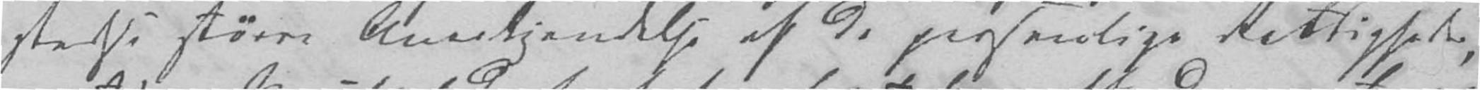stedse større Anerkjendelse af de personlige Rettigheder,
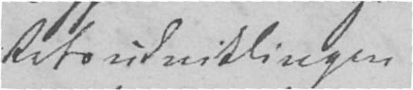Retsudviklingen
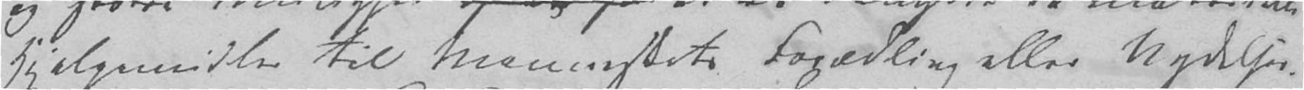Hjelpemidler til Menneskets Forædling eller Nydelser.
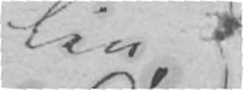Liv,
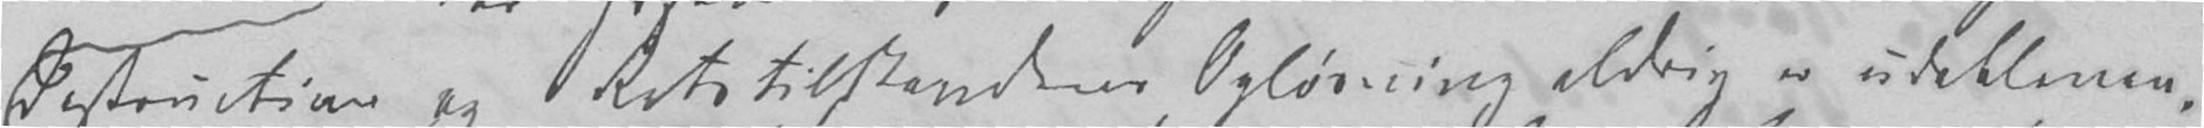Destruction og Retstilstandens Opløsning aldrig er udebleven,
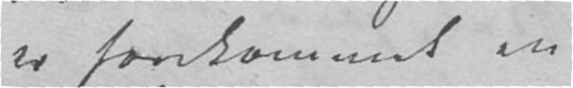er forekommet en
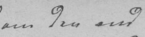om den end
